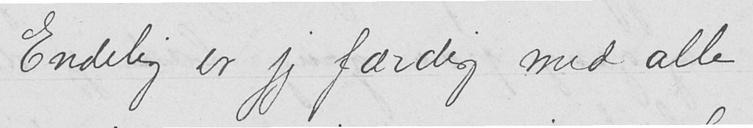Endelig er jeg færdig med alle
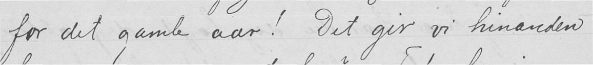for det gamle aar! Det gir vi hinanden
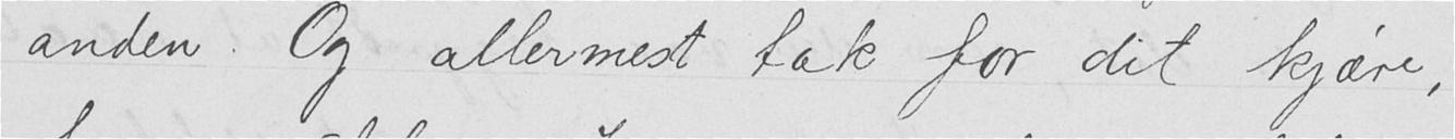anden. Og allermest tak for dit kjære,
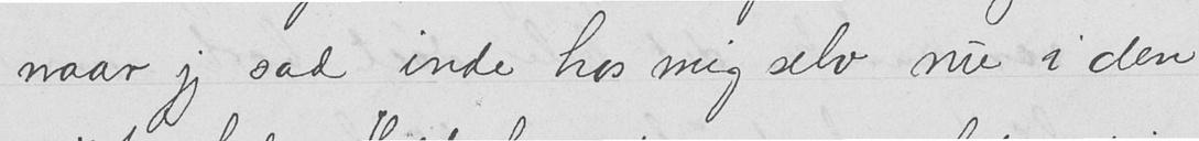naar jeg sad inde hos mig selv nu i den
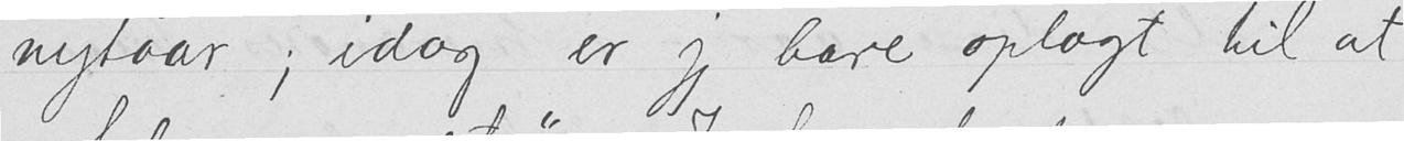nytaar; idag er jeg bare oplagt til at
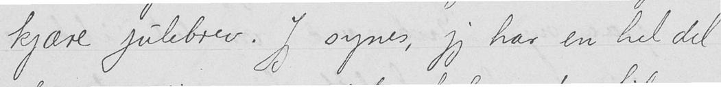kjære julebrev. Jeg synes, jeg har en hel del
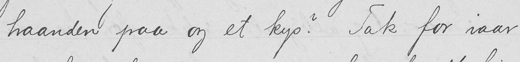haanden paa og et kys? Tak for iaar
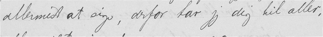allermest at sige, derfor tar jeg dig til aller,
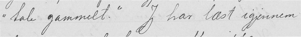"tale gammelt." Jeg har læst igjennem
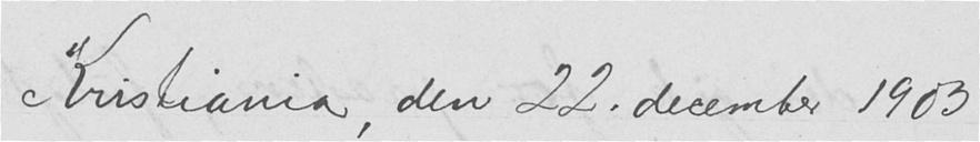Kristiania, den 22. december 1903
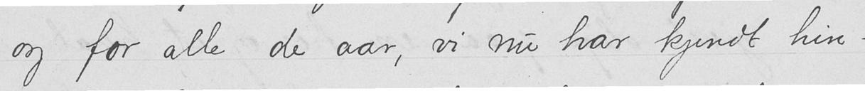og for alle de aar, vi nu har kjendt hin-
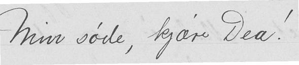Min søde, kjære Dea!
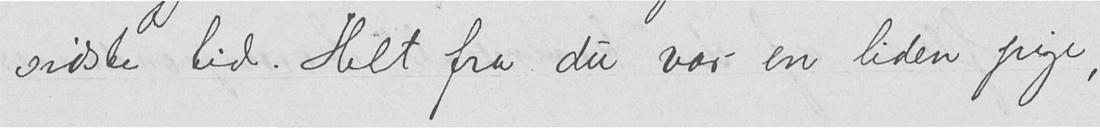sidste tid. Helt fra du var en liden pige,
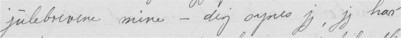julebrevene mine - dig synes jeg, jeg har
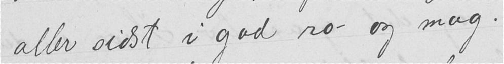aller sidst i god ro - og mag.
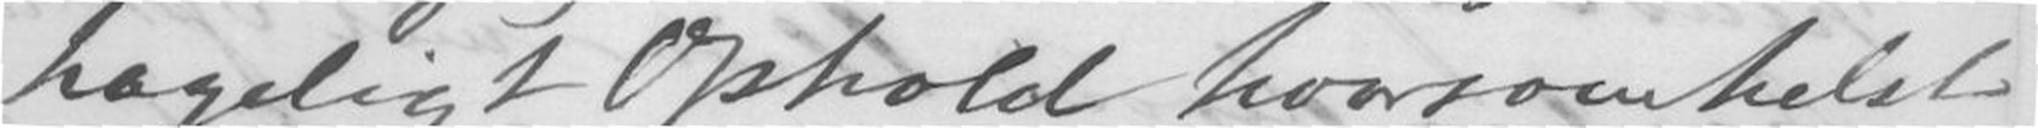hageligt Ophold hvorsomhelst
